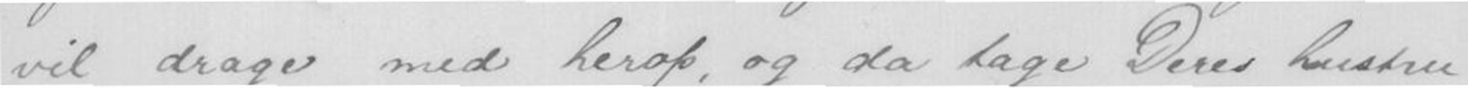vil drage med herop, og da tage Deres hustru
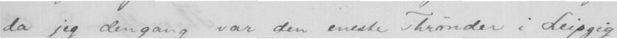da jeg dengang var den eneste Trønder i Leipzig
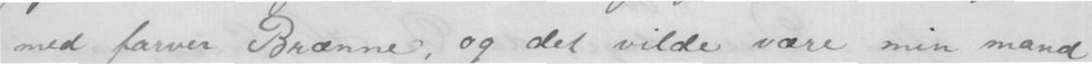med farver Brænne, og det vilde være min mand
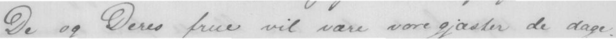De og Deres frue vil være vore gjæster de dage.
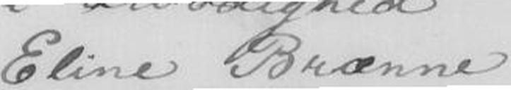Eline Brænne
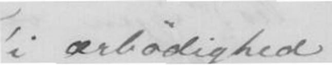i ærbødighed
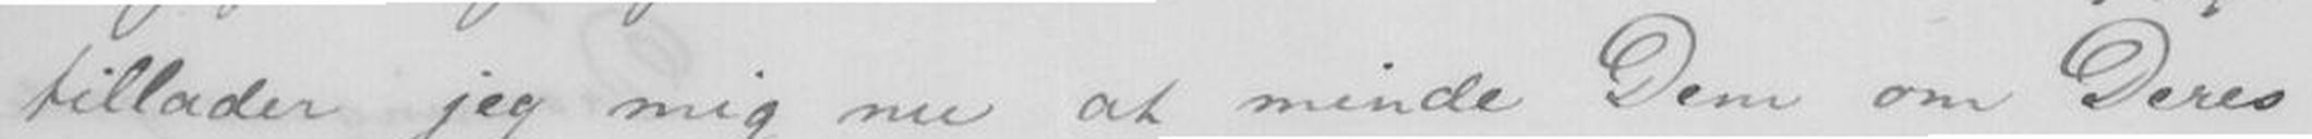tillader jeg mig nu at minde Dem om Deres
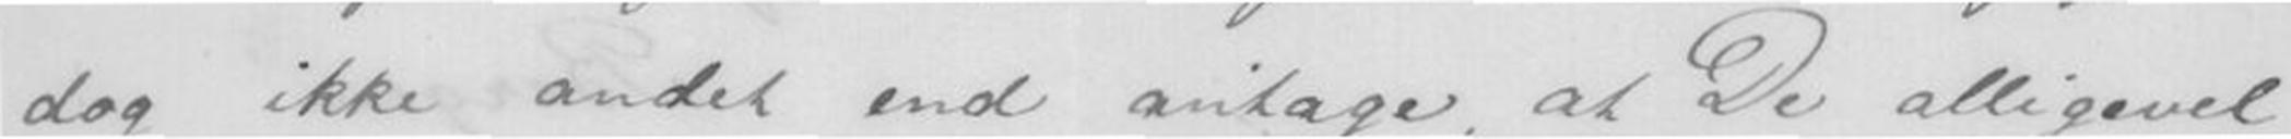dog ikke andet end antage, at De alligevel
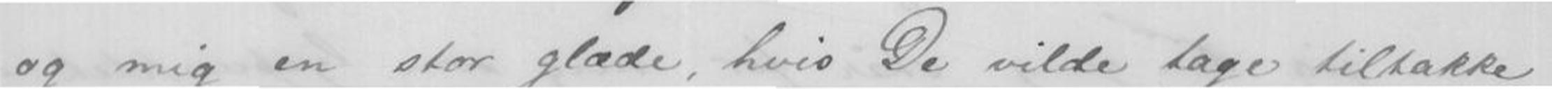og mig en stor glæde, hvis De vilde tage tiltakke
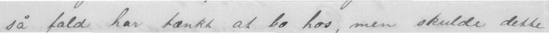så fald har tænkt at bo hos, men skulde dette
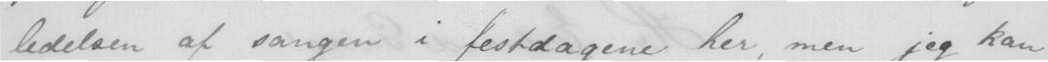ledelsen af sangen i festdagene her, men jeg kan
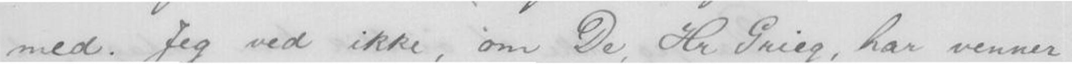med. Jeg ved ikke, om De, Hr Grieg, har venner
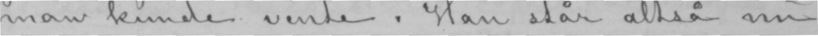man kunde vente. Han står altså nu
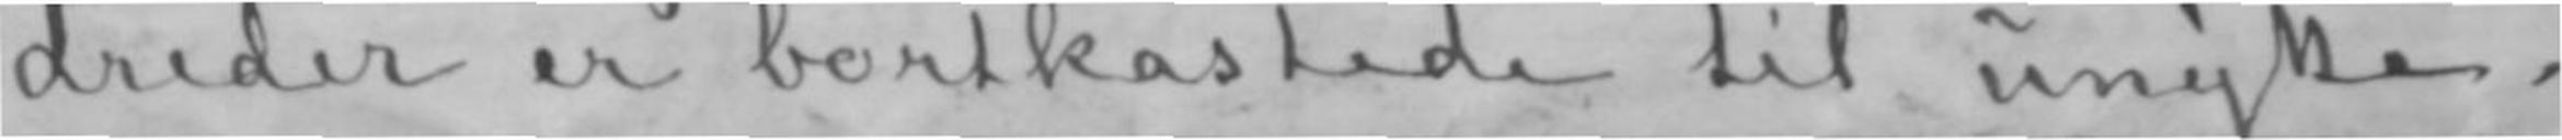dreder er bortkastede til unytte.
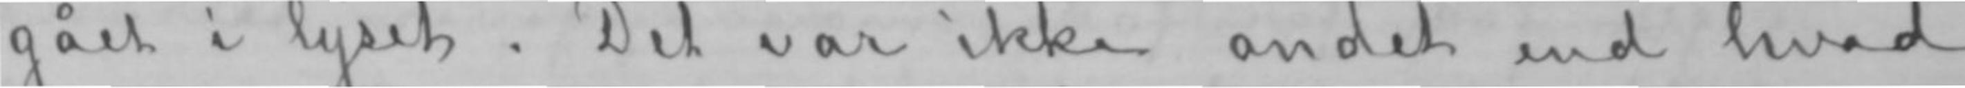gået i lyset. Det var ikke andet end hvad
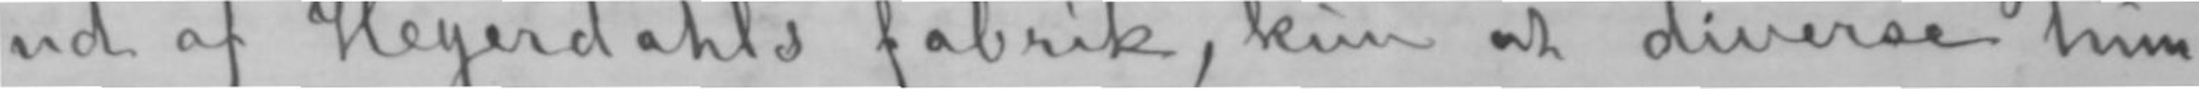ud af Heyerdahls fabrik, kun at diverse hun-
